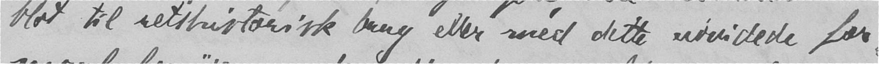blot til retshistorisk brug eller med dette udvidede for-
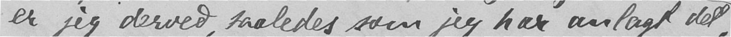er jeg derved, saaledes som jeg har anlagt det,
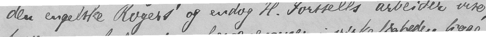den engelske Rogers' og endog H. Forssells arbeider vise,
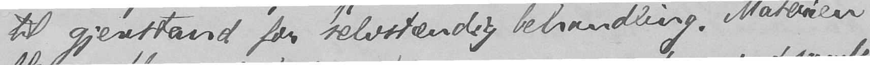til gjenstand for selvstændig behandling. Materien
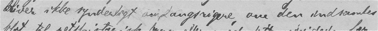bliver ikke synderligt omfangsrigere, om den indsamles
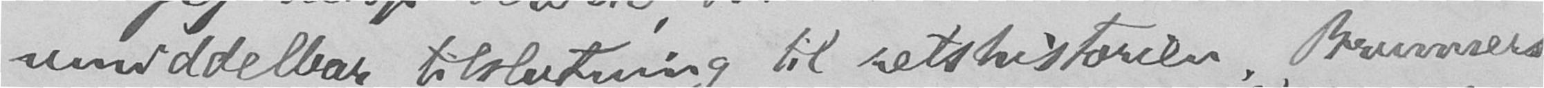umiddelbar tilslutning til retshistorien. Brunners
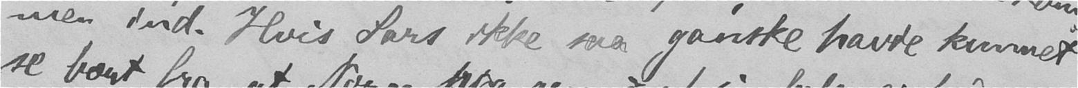men ind. Hvis Sars ikke saa ganske havde kunnet
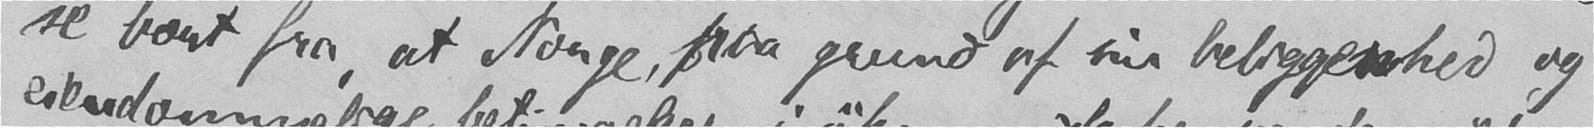se bort fra, at Norge, paa grund af sin beliggenhed og
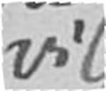vil
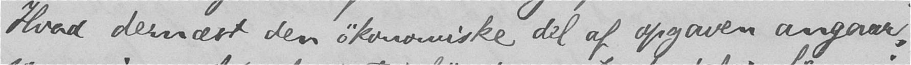Hvad dernæst den økonomiske del af opgaven angaar,
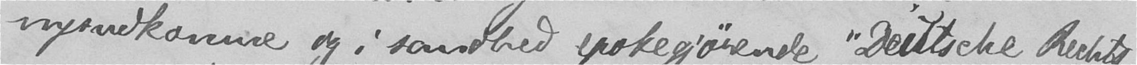nys udkomne og i sandhed epokegjørende "Deutsche Rechts-
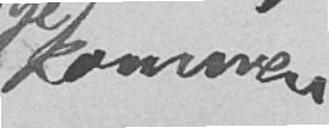kommen
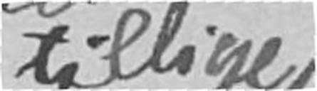tillige
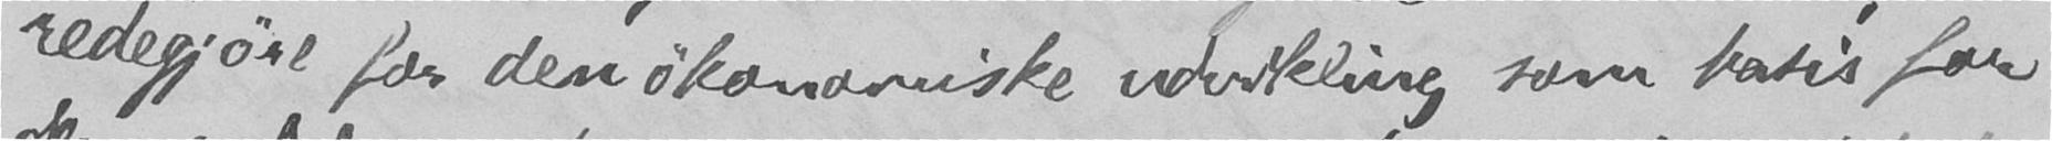redegjøre for den økonomiske udvikling som basis for
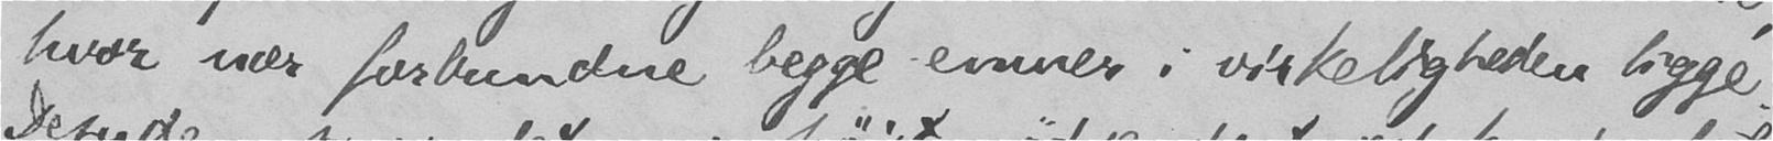hvor nær forbundne begge emner i virkeligheden ligge.
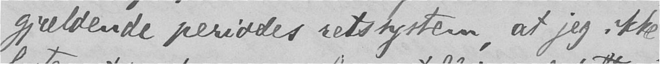gjældende periodes retssystem, at jeg ikke
